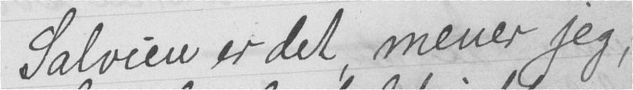Salvien er det mener jeg,
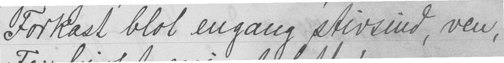Forkast blot engang stivsind, ven,
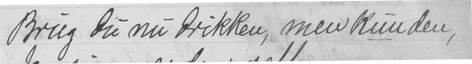Brug du nu drikken, men kun den,
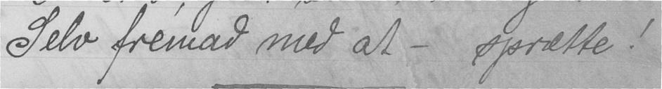Selv fremad med at - sprætte!
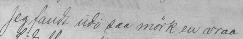Jeg fandt udi saa mørk en vraa
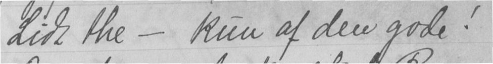Lidt the - kun af den gode!
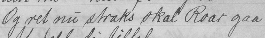Og ret nu straks skal Roar gaa
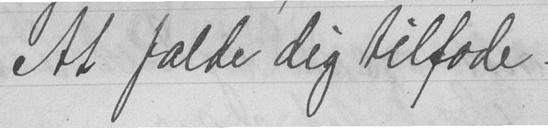At falde dig tilfode.
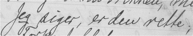Jeg siger, er den rette;
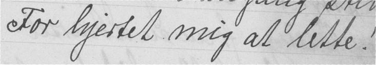For hjertet mig at lette!
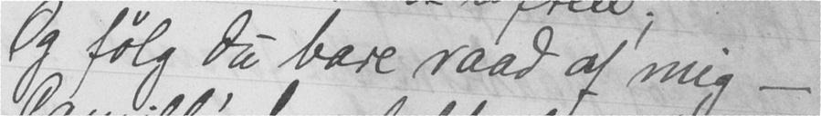Og følg du bare raad af mig -
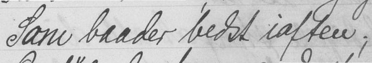Som baader bedst iaften;
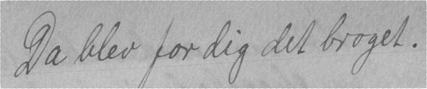Da blev for dig det broget.
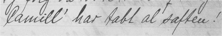Camill' har tabt al saften!
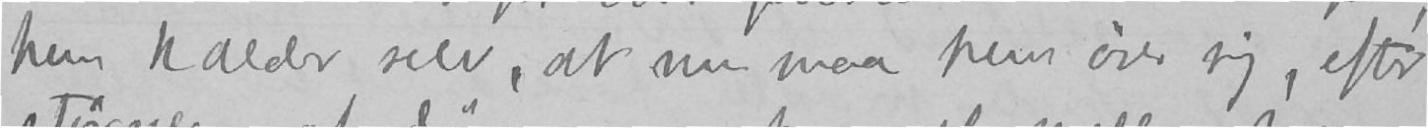hun kalder selv, at nu maa hun øve sig, efter
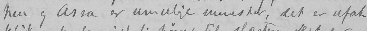hun og Assa er umulige mennesker, det er ufat-
